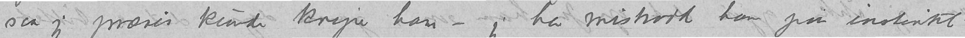saa jeg præcis kunde <knipe> ham – jeg har mistrodd ham paa instinkt.
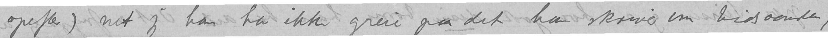opefter) vet jeg han har ikke greie paa det han skriver om tidsaanden,
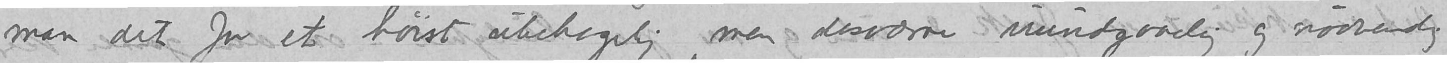man det for et høist ubehagelig, men desværre uundgaaelig og nødvendig
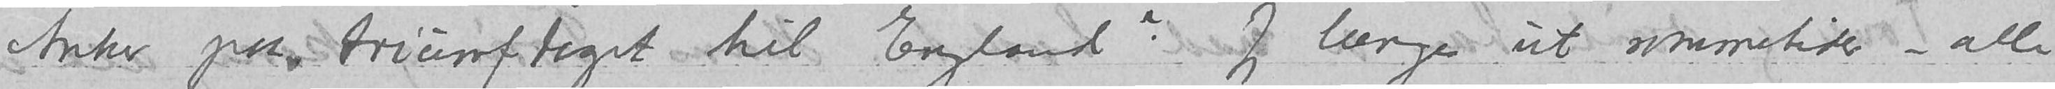Anker paa triumftoget til England? Jeg længes ut sommetider – alle
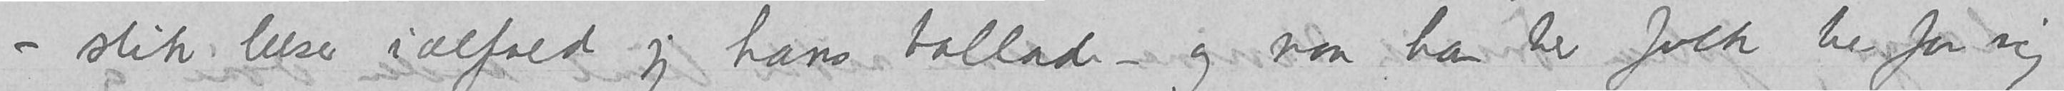– slik læser ialfald jeg hans ballade – og naar han ber folk be for sig
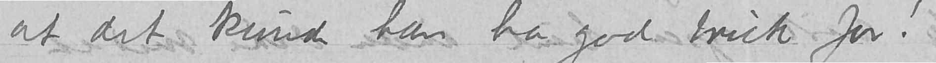at det kunde han ha god bruk for!
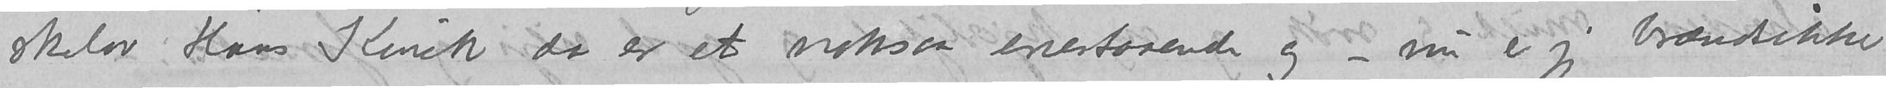skelov Hans Kinck da er et noksaa enestaaende og – nu er jeg brændsikker
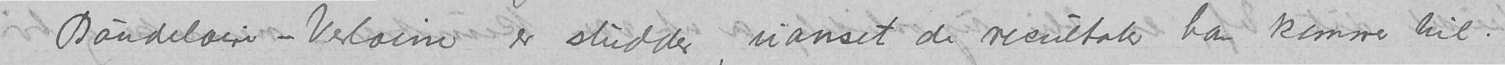Baudelaire-Verlaine er sludder, uanset de resultater han kommer til.
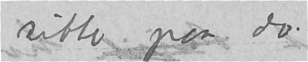sitter paa do.
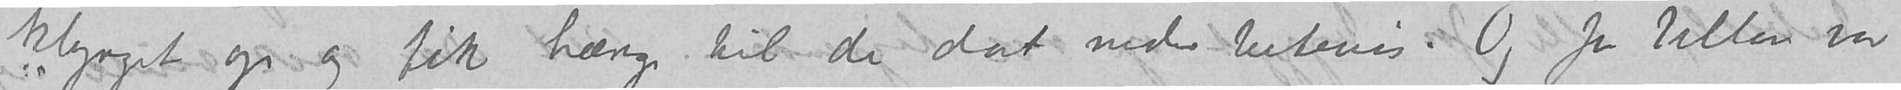klynget op og fik hænge til de dat nedover lutenvis. Og for Villon var
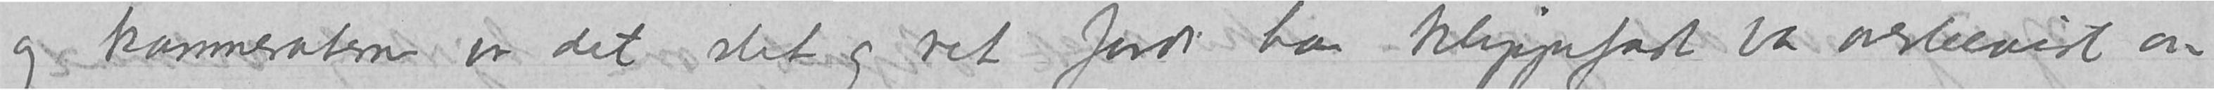og kammeraterne er det slet og ret fordi han klippefast var overbevist om
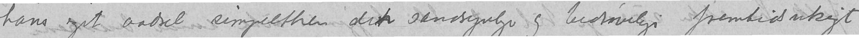hans eget aadsel simpelthen den sandsynelige og bedrøvelige fremtidsutsigt
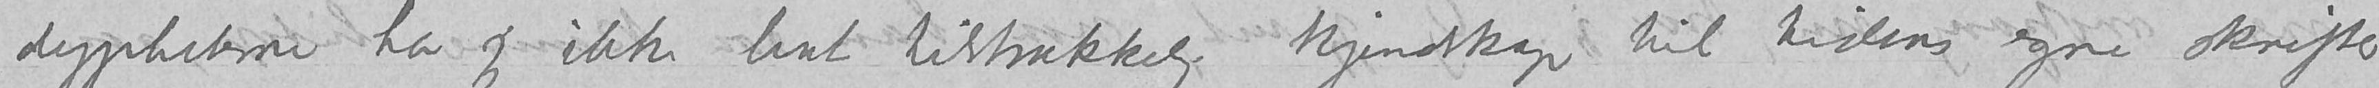dypheterne har jeg ikke hat tilstrækkelig kjendskap til tidens egne skrifter
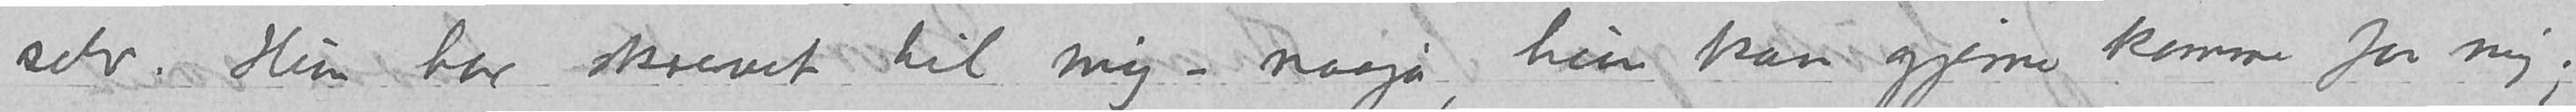selv. Hun har skrevet til mig – naaja, hun kan gjerne komme for mig;
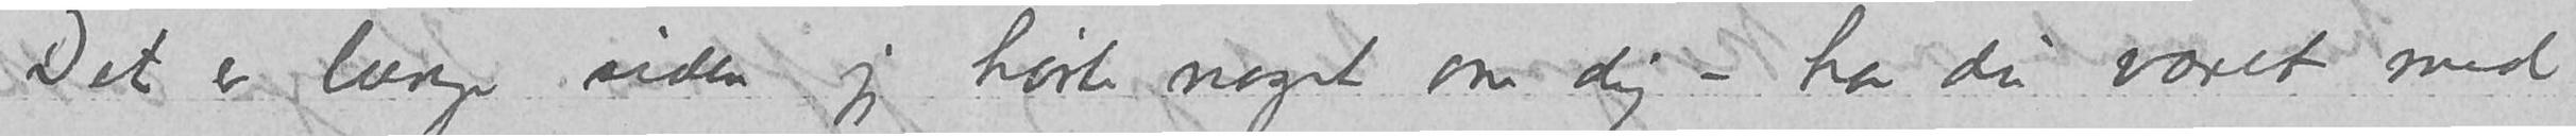Det er længe siden jeg hørte noget om dig – har du været med
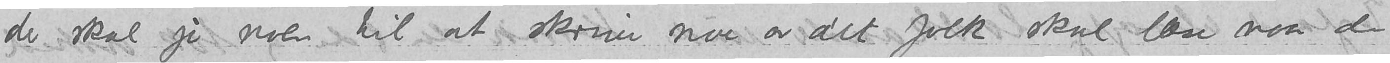der skal jo noen til at skrive noe av det folk skal læse naar de
